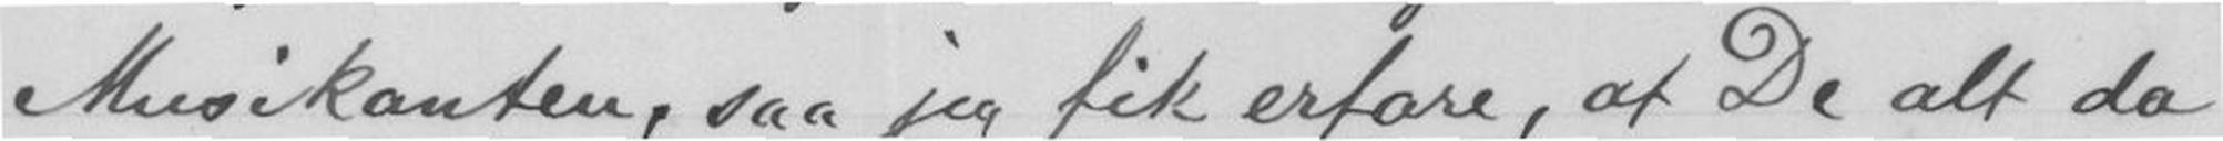Musikanten, saa jeg fik erfare, at De alt da
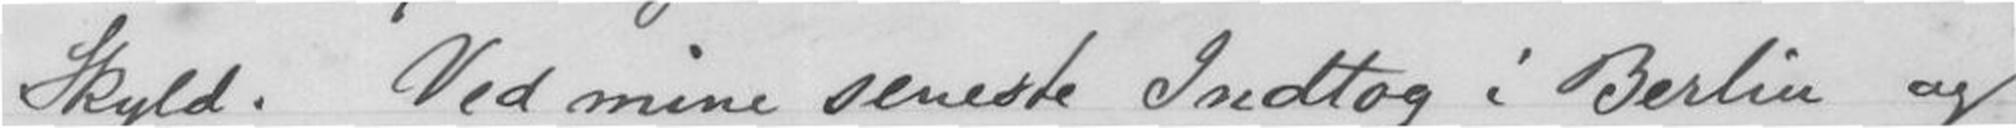Skyld. Ved mine seneste Indtog i Berlin og
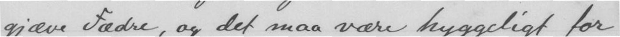gjæve Fædre, og det maa være hyggeligt for
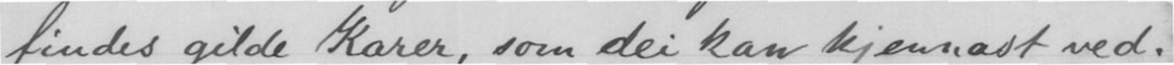findes gilde Karer, som dei kan kjennast ved.
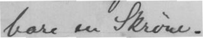bare en Skrøne.
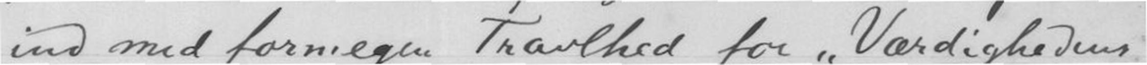ind med formegen Travhed for Værdighedens
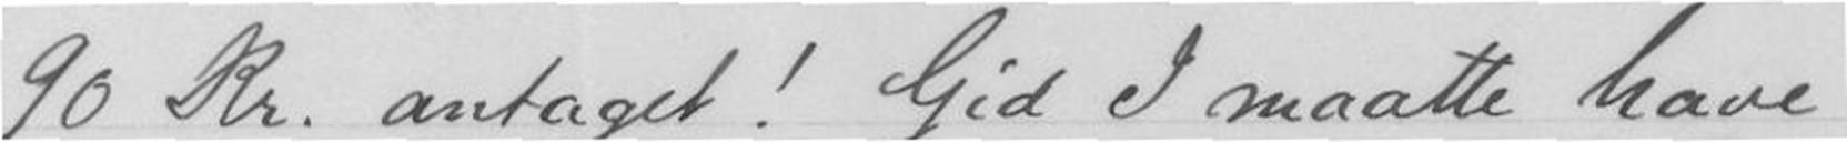90 Kr. antaget! Gid I maatte have
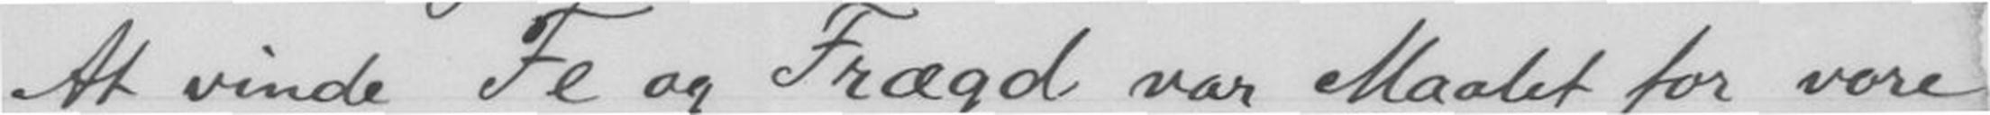At vinde Fe og Frægd var Maalet for vore
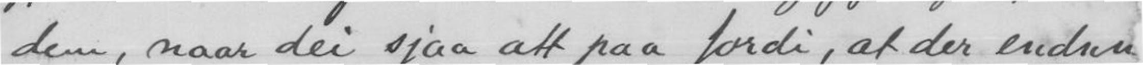dem, naar dei sjaa att paa fordi, at der endnu
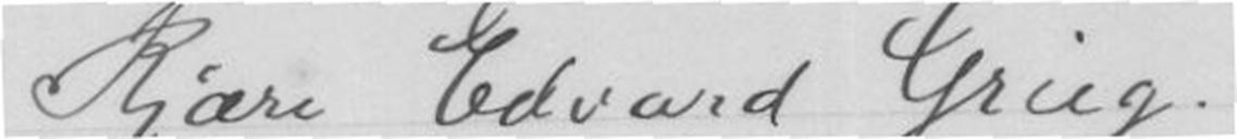Kjære Edvard Grieg.
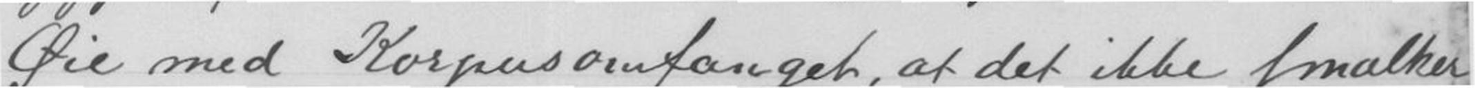Øie med Korpusomfanget, at det ikke smælter
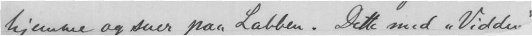hjemme og suer paa Labben. Dette med "Vidde
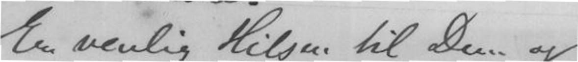En venlig Hilsen til Dem og
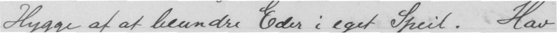Hygge af at beundre Eder i eget Speil. Hav
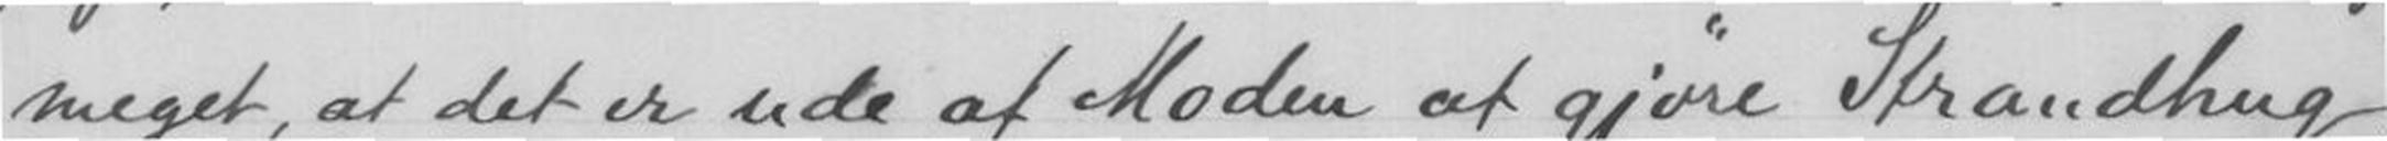meget, at det er ude af Moden at gjøre Strandhug
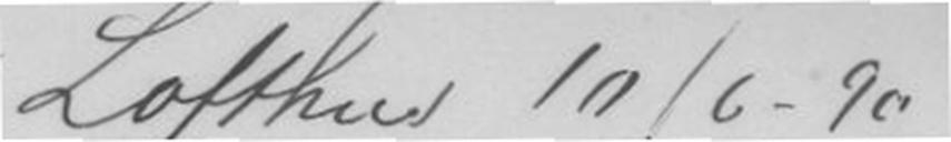Lofthus 10/6-90
